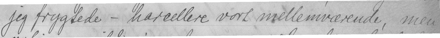jeg frygtede - harcellere vort mellemværende, men
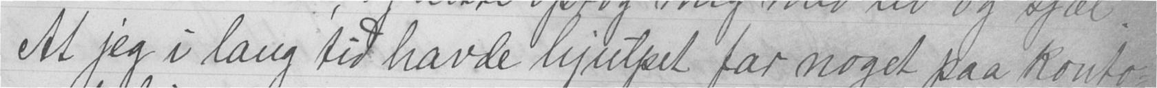At jeg i lang tid havde hjulpet far noget paa konto-
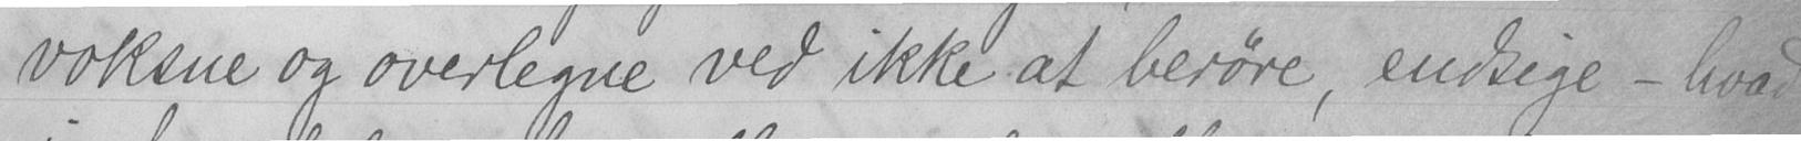voksne og overlegne ved ikke at berøre, endsige - hvad
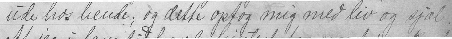ude hos hende; og dette optog mig med liv og sjæl.
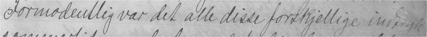Formodentlig var det alle disse forskjellige indtryk
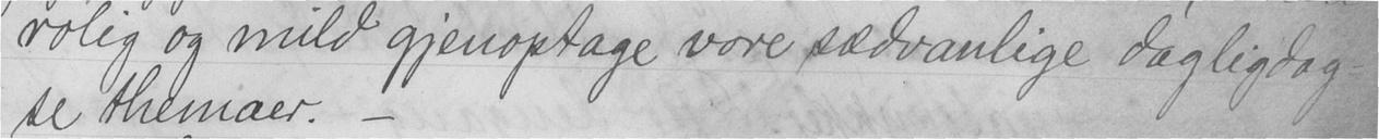rolig og mild gjenoptage være sædvanlige dagligdag-
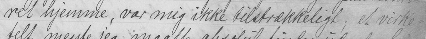ret hjemme, var mig ikke tilstrækkeligt; et virke-
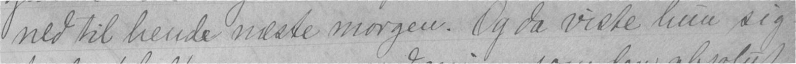ned til hende næste morgen. Og da viste hun sig
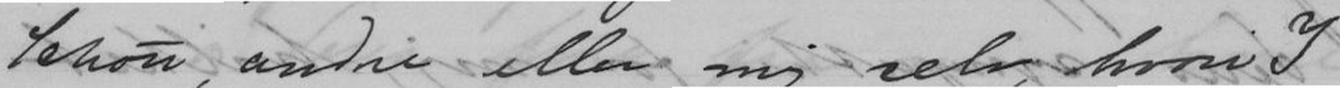Schou, andre eller mig selv, hvori I
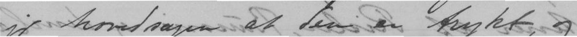jo hovedsagen at den er trykt, og
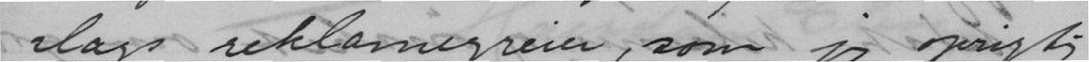slags reklamegreier, som jeg oprigtig
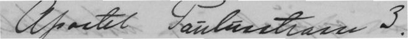Apostel Paulusstrasse 3.
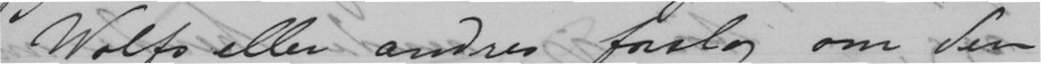Wolfs eller andres forslag om den
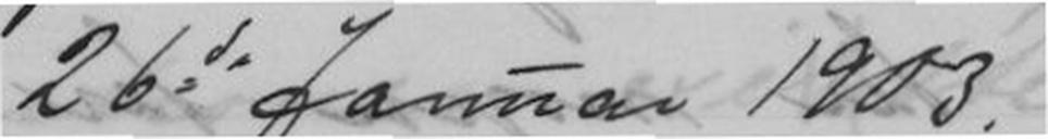26dejanuar 1903.
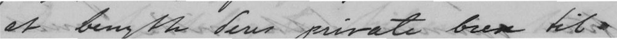at benytte deres private breve til
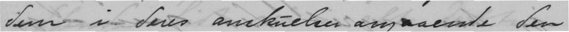dem i deres anskuelser angaaende den
